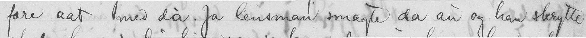fare aat med da. Ja lensman smagte da an og han skrytte
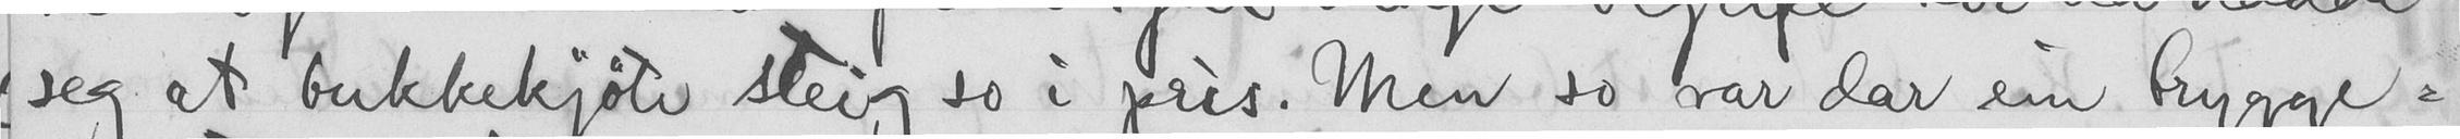seg at bukkekjøte steig so i pris. Men so var dar ein brygge-
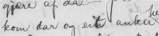kom dar og eit anker
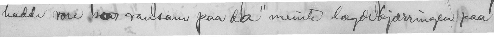hadde vore so vansam paa da" meinte lægdekjærringen paa
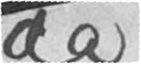da
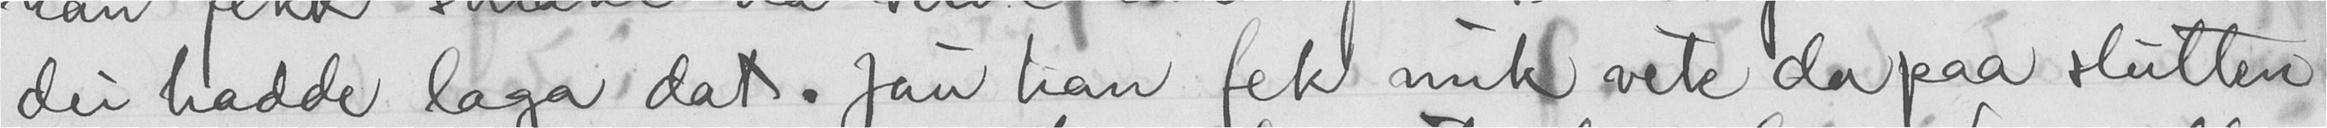dei hadde laga dat. Jau han fek nuk vete dapaa slutten
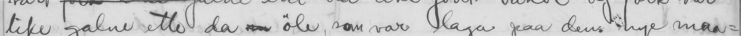like galne ette da  øle, som var laga paa den nye maa-
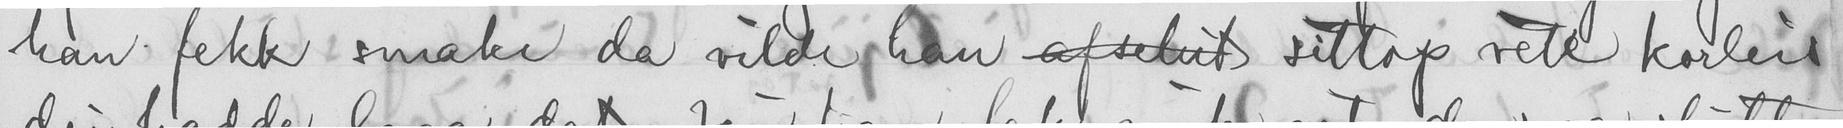han fekk smake da vilde han  sittop vete korleis
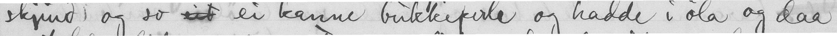skjind og so  ei kanne bukkeperle og hadde i øla og daa
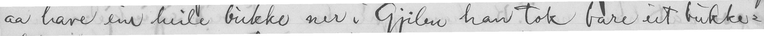aa have ein heile bukke ner i Gjilen han tok bare ut bukke-
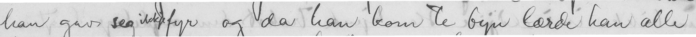han gav seg ikkje fyr og da han kom te byn lærde han alle
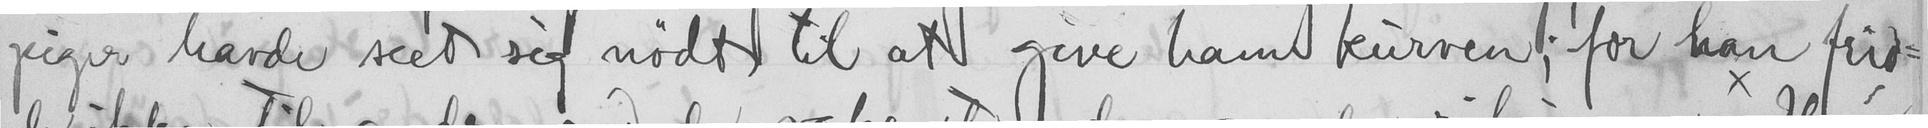piger havde seet sig nødt til at give ham kurven; for han frid-
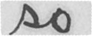so
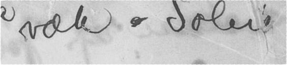væk. Solen
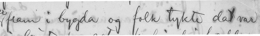fram i bygda og folk tykte dat var
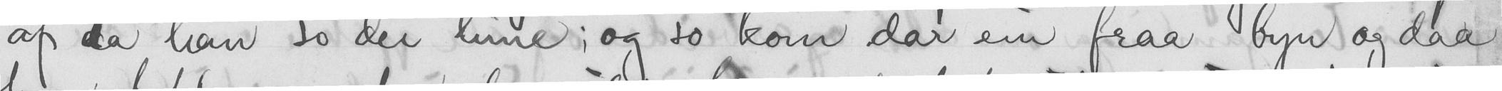af da han so dei hine; og so kom dar ein fraa byn, og daa
